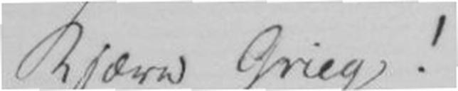Kjære Grieg!
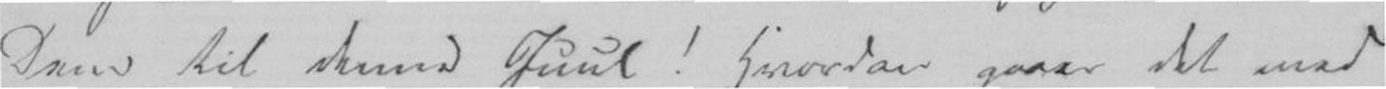Vem til denne Juul! Hvordan gaaer det med
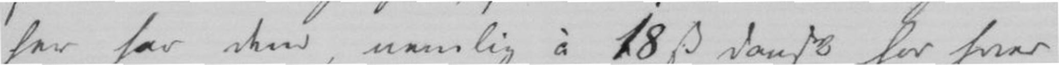her har dem, nemlig á 18 ss dansk for hver
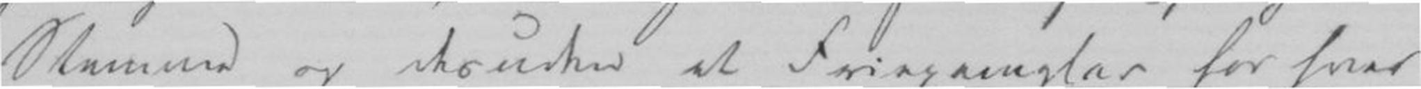Stemme og desuden et Friexemplar for hver
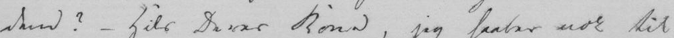dem? - Hils Deres Kone, jeg haaber nok til
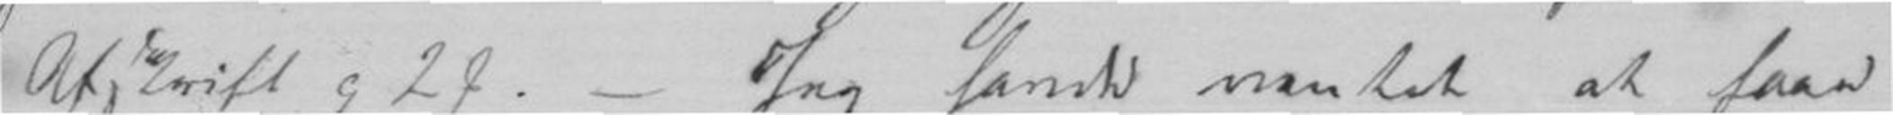Afskrift c 2 p - Jeg havde ventet at faae
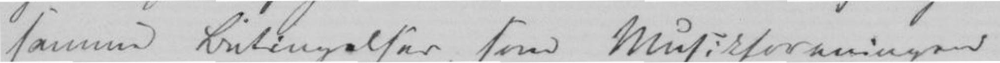samme Betingelser, som Musikforeningen
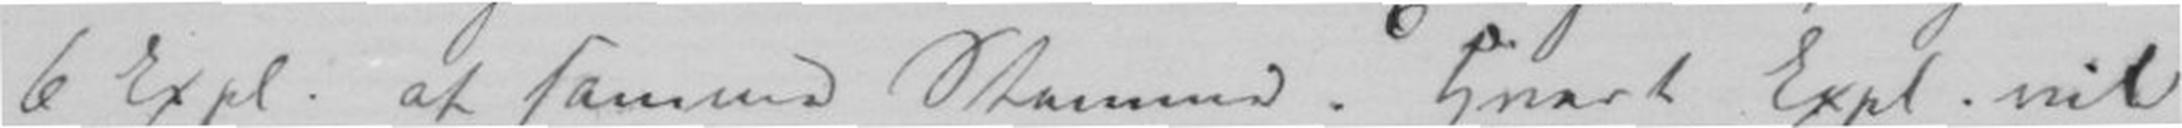& Expl. af samme Stemme. Hvert Expl. vil
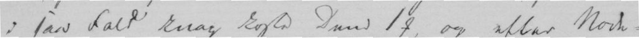i saa Fald knap koste Dem 1 p og efter Node-
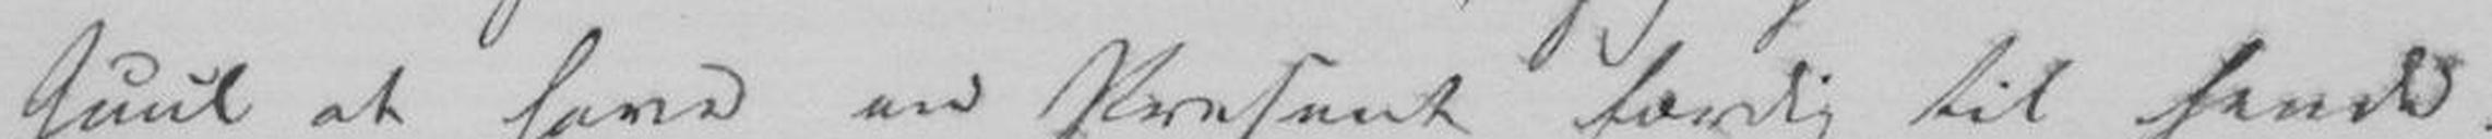Juul at have en Present Færdig til hende.
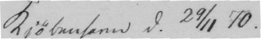Kjøbenhavn d. 29/11 70.
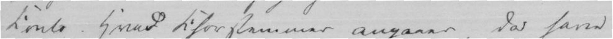Konto. Hvad Chorstemmer angaar, de have
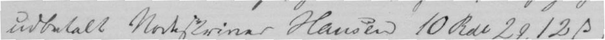udbetalt Nodskriver Hansen 10 Rdl 2 p 12 ss,
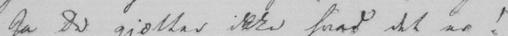Ja De gjætter ikke hvad der er!
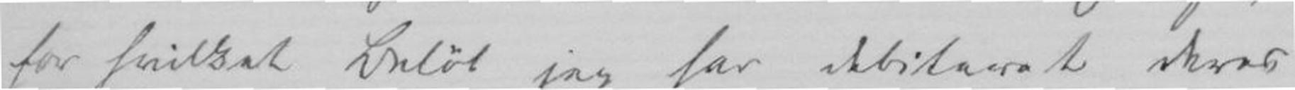for hvilket Beløb jeg har debiteret deres
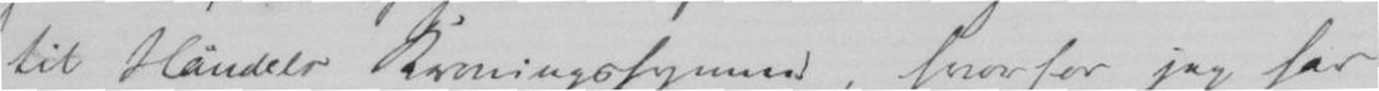til Händels Kroningshymne, hvorfor jeg har
